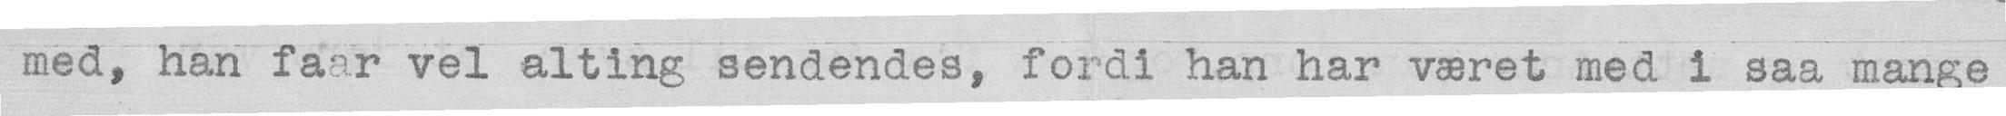med, han faar vel alting sendendes, fordi han har været med i saa mange
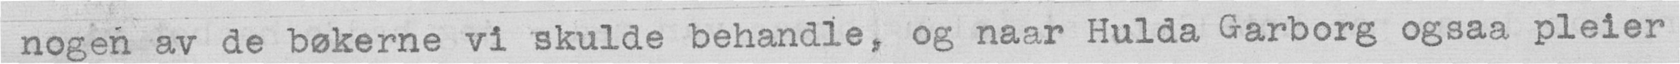nogen av de bøkerne vi skulde behandle, og naar Hulda Garborg ogsaa pleier
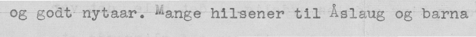og godt nytaar. Mange hilsener til Åslaug og barna
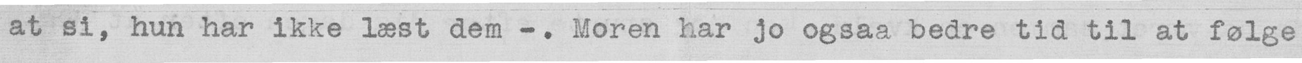at si, hun har ikke læst dem -. Moren har jo ogsaa bedre tid til at følge
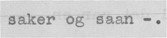saker og saan -.
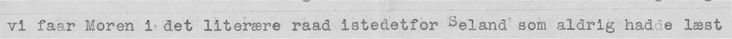vi faar Moren i det literære raad istedetfor Seland som aldrig hadde læst
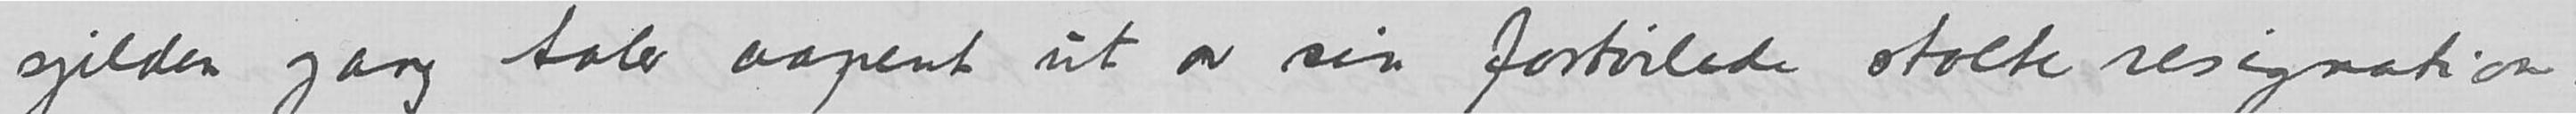sjelden gang taler aapent ut av sin fortvilede stolte resignation.
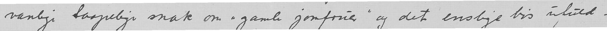vanlige taapelige snak om «gamle jomfruer» og det enslige livs ufuld
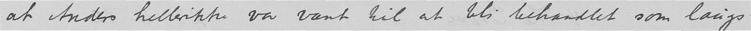at Anders hellerikke var vant til at bli behandlet som laugs-
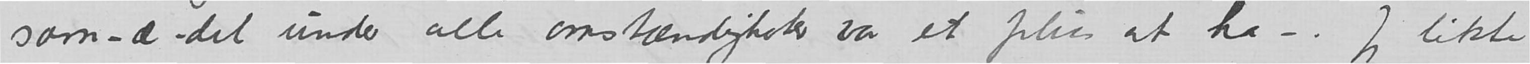som - æ - det under alle omstændigheter var et plus at ha -. Jeg likte
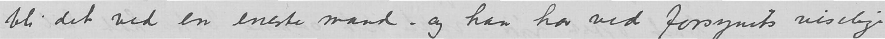bli det ved en eneste mand. - og han har ved forsynets viselige
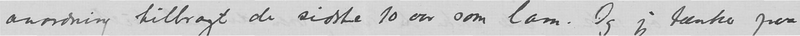anordning tilbragt de sidste 10 aar som lam. Og jeg tænker paa
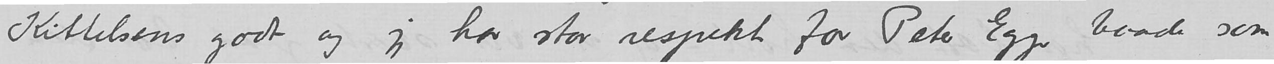Kittelsens godt og jeg har stor respekt for Peter Egge baade som
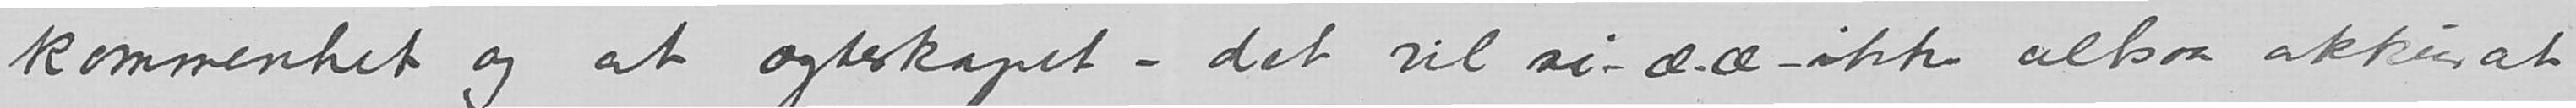kommenhet og at ægteskapet - det vil si - æ-æ - ikke altsaa ak
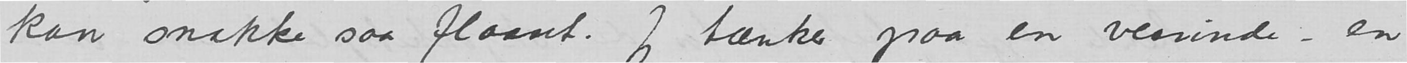kan snakke saa flaaset. Jeg tænker paa en venninde - en
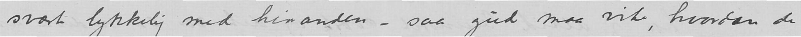svært lykkelig med hinanden - saa gud maa vite, hvordan de
